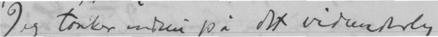Jeg tænker endnu på det vidunderlig
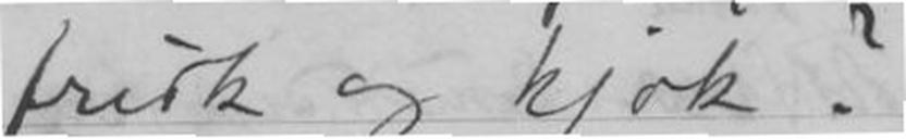frisk og kjæk?
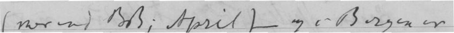(mer end BB; April) - og i Bergen er
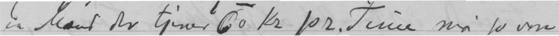en Mand der tjener To Kr pr. Time må jo være
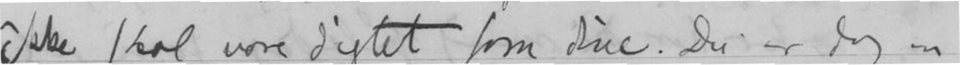ikke skal være digtet som dine. Du er dog en
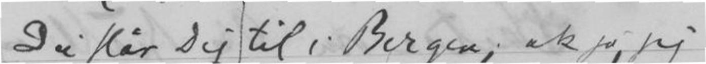Du står Dig til Bergen, ak ja, jeg
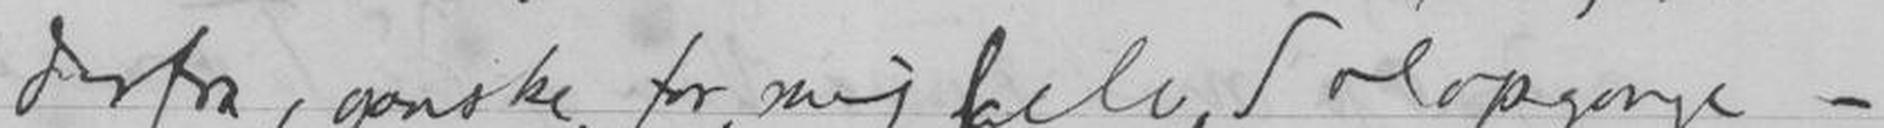jeg har derfra, ganske for mig selv, Solopgange -
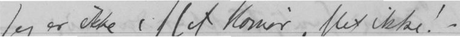Jeg er ikke i slet Homør. slet ikke! -
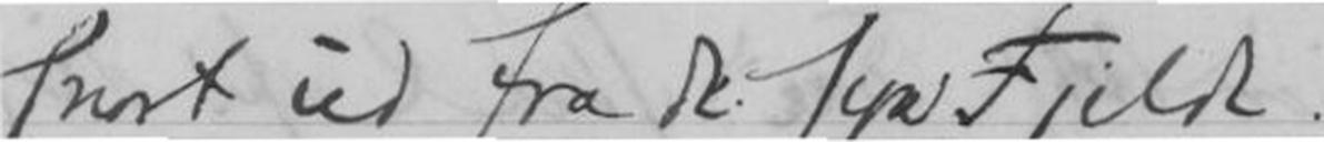snart ud fra de syvn Fjelde!
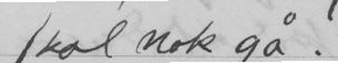skal nok gå!
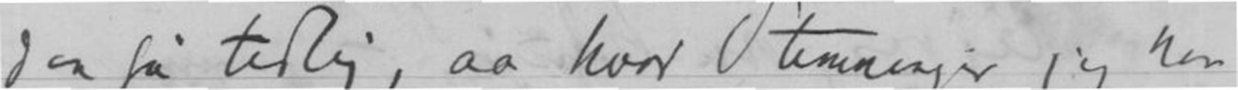den så tidlig, aa hvor Stemninger
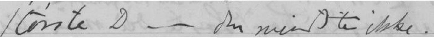største D - den mindste ikke.
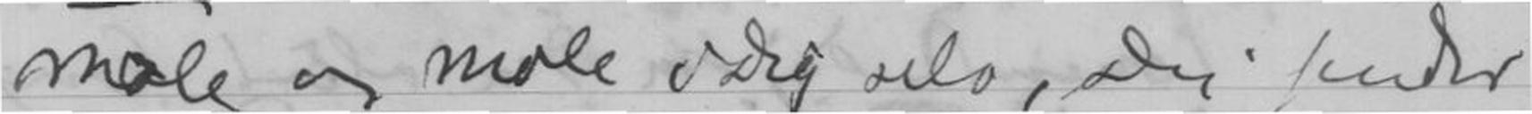male og male i Dig selv, Dig sender
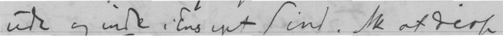ude og inde i Ens eget Sind. Ak at disse
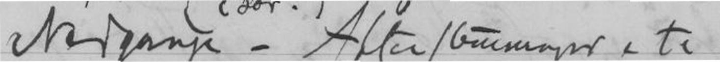Nedgange - Aften stemninger e tc
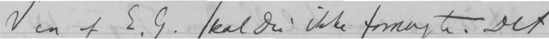Ven af E.G. skal du ikke fornægte. Det
